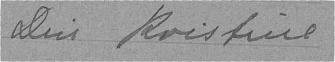Din Kristine.
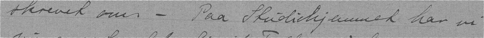skrevet om - Paa Studiehjemmet har vi
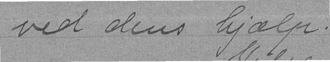ved deres hjælp.
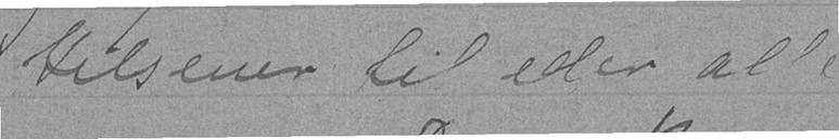Hilsener til eder alle
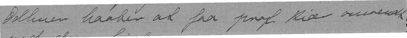Odhner haaber at faa prof. Kiær omvendt
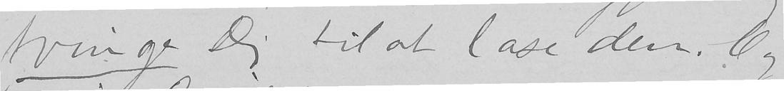tvinge Dig til at læse den. Og
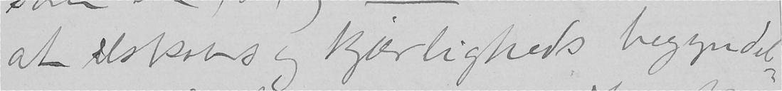at elskovs og kjærligheds begyndel-
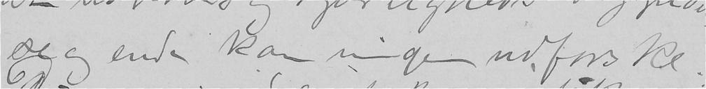se og ende kan ingen udforske.
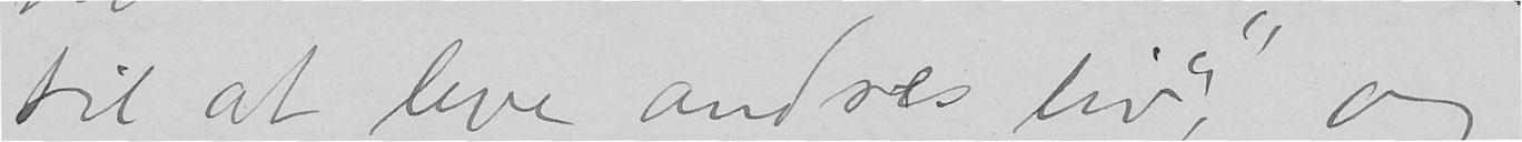til at leve andres liv" og
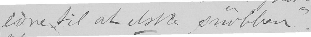évne til at elske snobben"
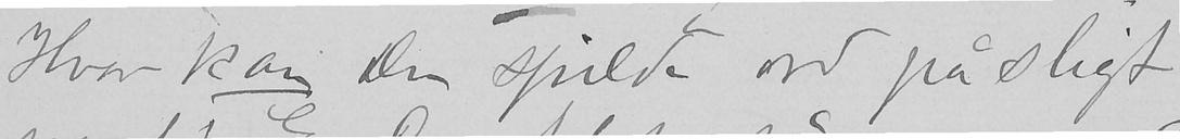Hvor kan Du spilde ord på sligt
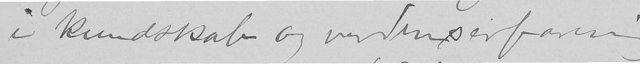i kundskab og verdenserfaring
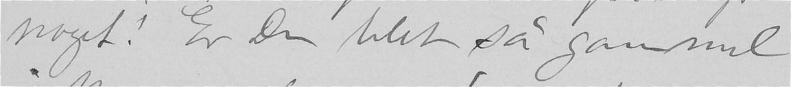noget! Er Du blit så gammel
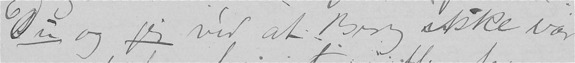Du og jeg véd at Berg ikke var
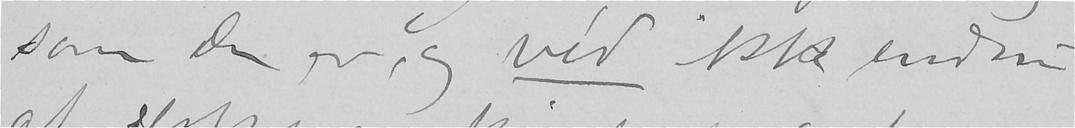som Du er, og véd ikke endnu
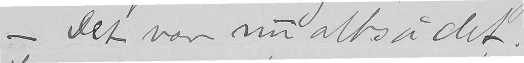- Det var nu altså det.
